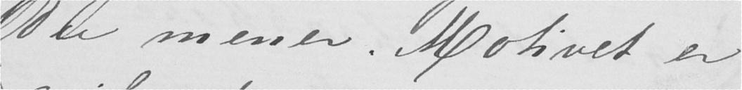Du mener. Motivet er
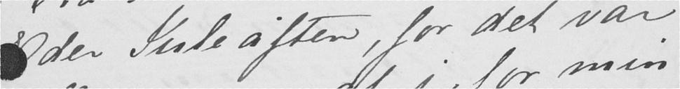Eder Juleaften, for det var
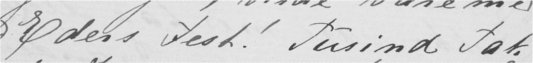Eders Fest! Tusind Tak
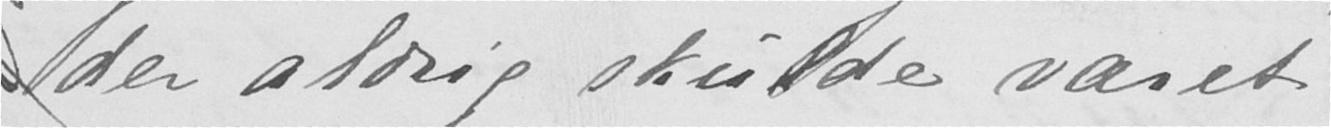der aldrig skulde været
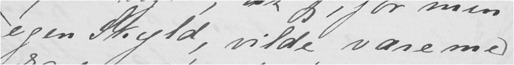egen Skyld, vilde være med
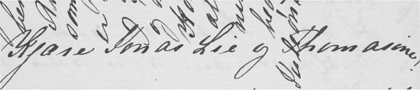Kjære Jonas Lie og Thomasine!
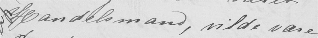Handelsmand, vilde være
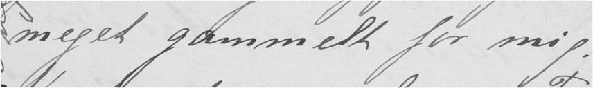meget gammelt for mig.
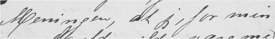Meningen, at jeg, for min
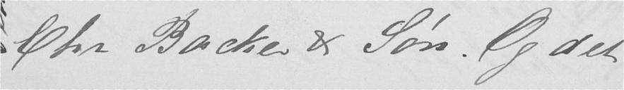Chr Backer & Søn. Og det
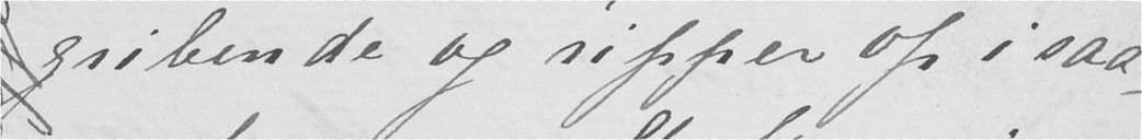gribende og ripper op i saa
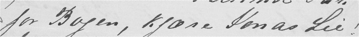for Bogen, kjære Jonas Lie!
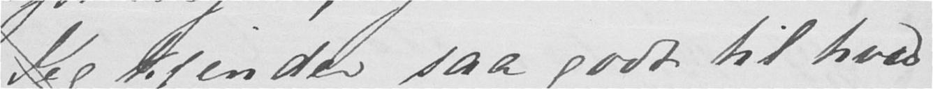Jeg kjender saa godt til hvad
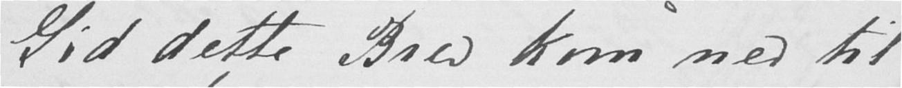Gid dette Brev kom ned til
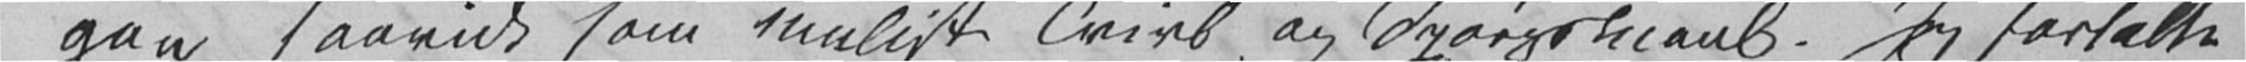gaa saavidt som muligt Tvivl og Spørgsmaal. Jeg fortalte
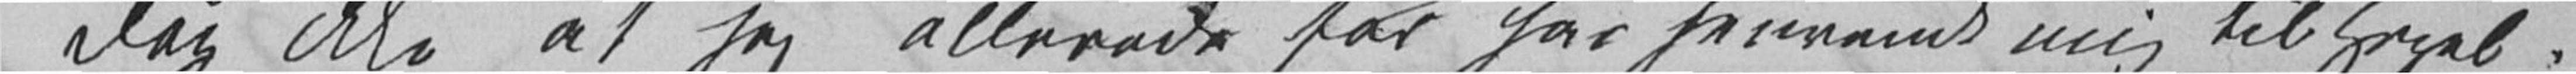dog ikke at jeg allerede før har henvendt mig til Hegel,
